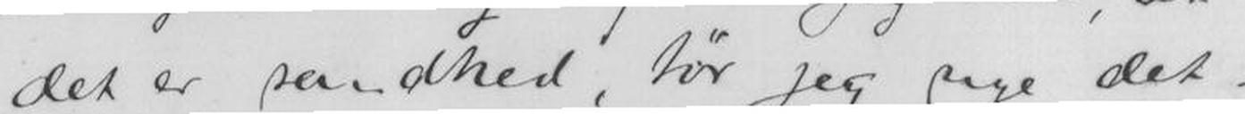det er sandhed, tør jeg sige det -
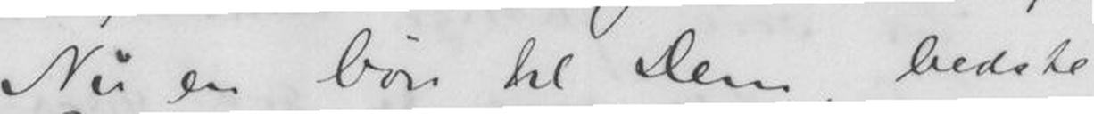Nu en bøn til Dem, bedste
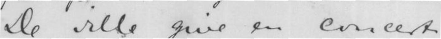De ville give en concert
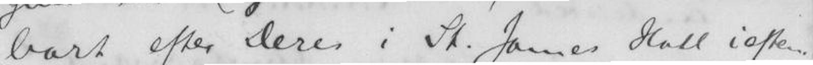bart efter Deres i St. James Hall iaften.
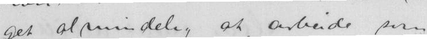get almindelig at arbeide som
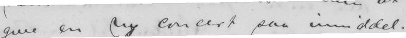give en ny concert saa umiddel-
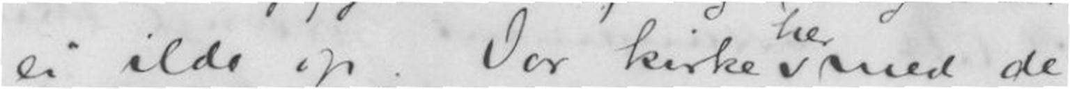ei ilde op. Vor kirke her med de
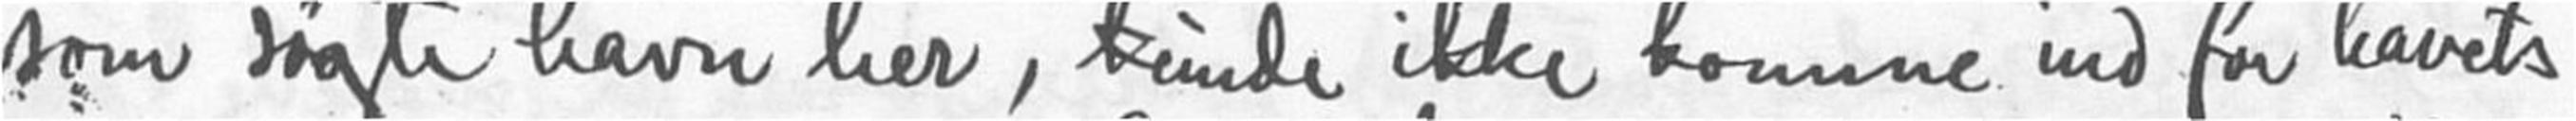som søgte havn her, kunde ikke komme ind for havets
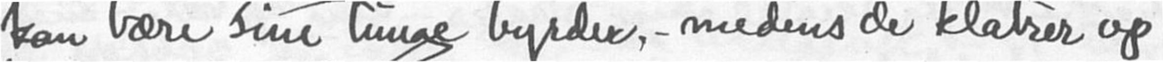kan bære sine tunge byrder, - medens de klatrer op
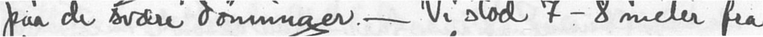paa de svære dønninger. - Vi stod 7-8 meter fra
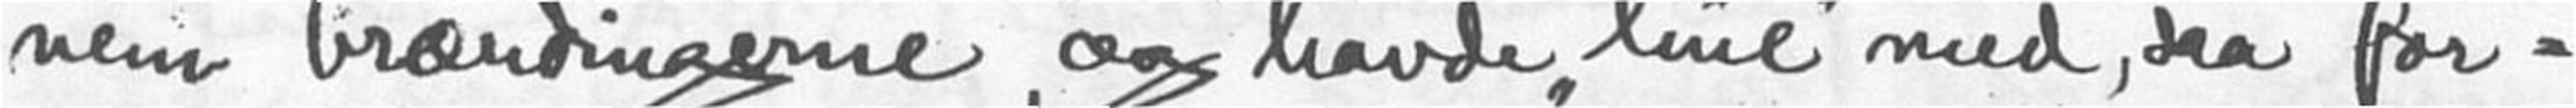nem brændingerne og havde "line" med, saa for-
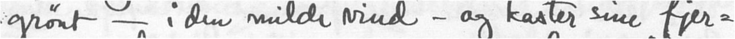grønt - i den milde vind - og kaster sine fjer-
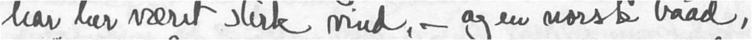har her været sterk vind, - og en norsk baad,
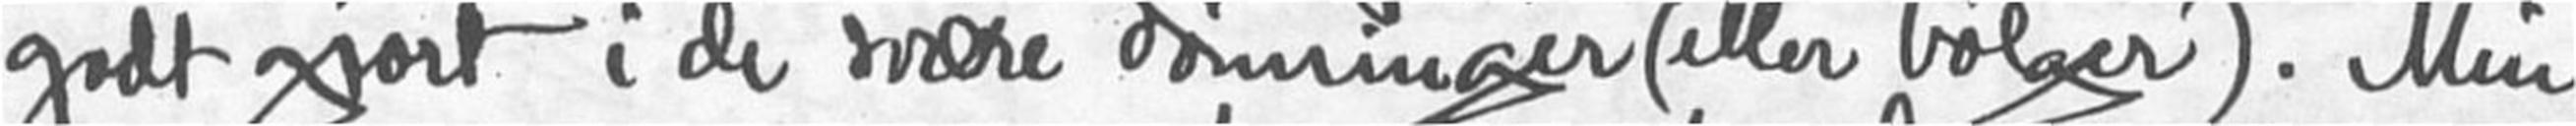godt gjort i de svære dønninger (eller bølger). Min
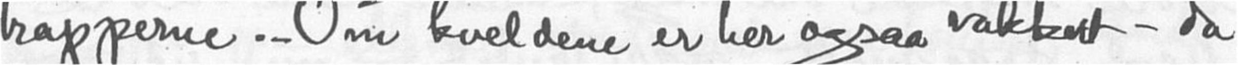trapperne. - Om kveldene er her ogsaa vakkert - da
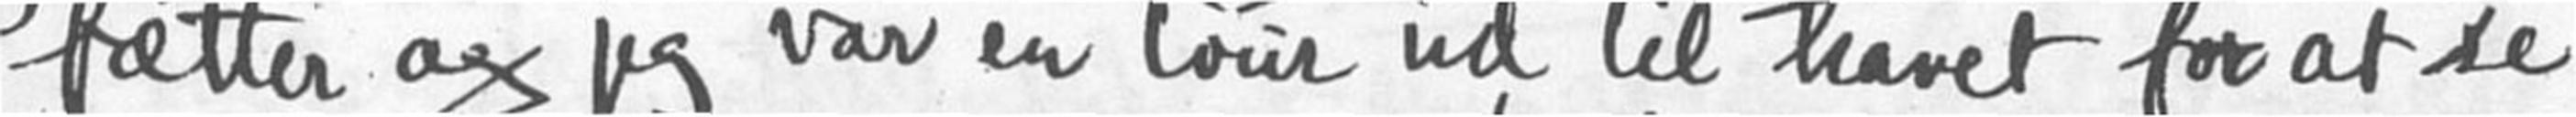fætter og jeg var en tour ud til havet for at se
